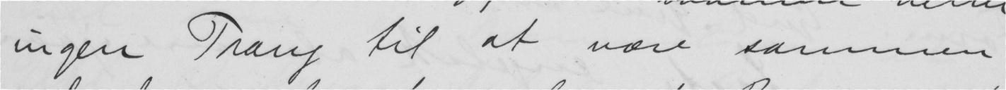ingen Trang til at være sammen
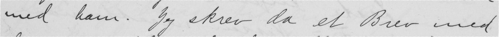med ham. Jeg skrev da et Brev med
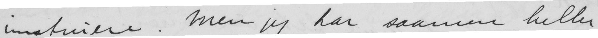instruere. Men jeg har saamen heller
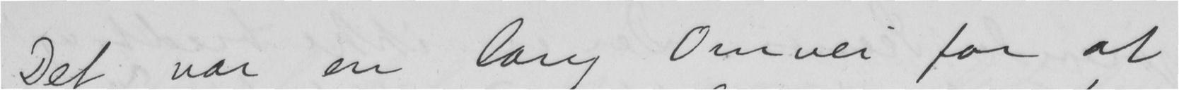Det var en lang Omvei for at
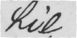Lie
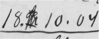18.10.04
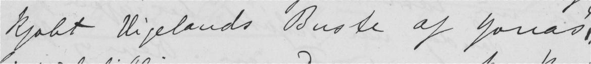kjøbt Vigelands Buste af Jonas
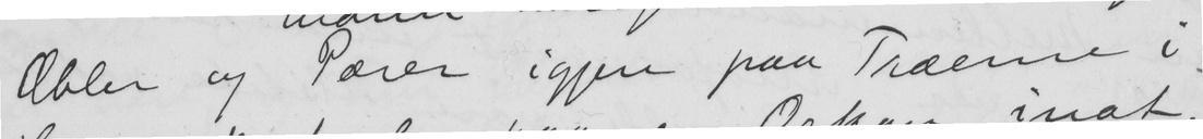Æbler og Pærer igjen paa Træerne i
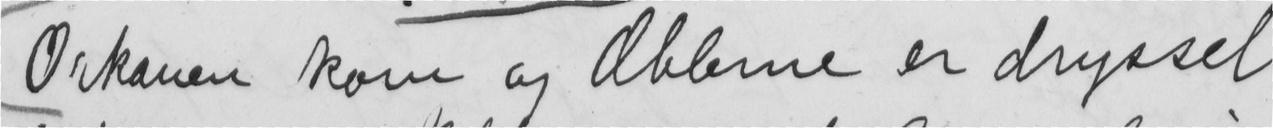Orkanen kom og Æblerne er drysset
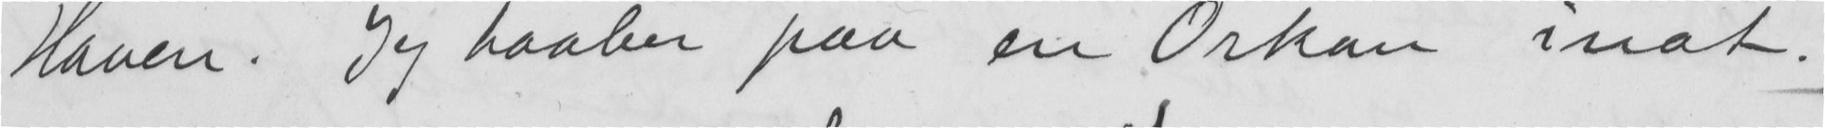Haven. Jeg haaber paa en Orkan inat.
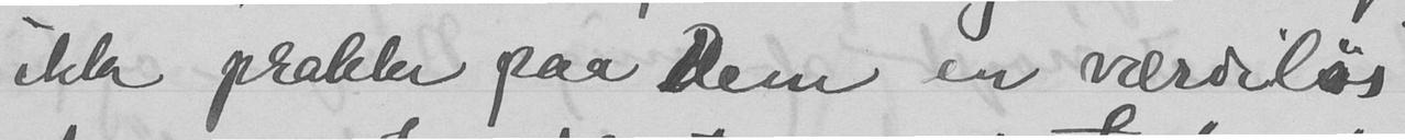ikke prakke paa Dem en værdiløs
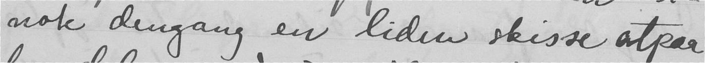nok dengang en liden skisse atpaa
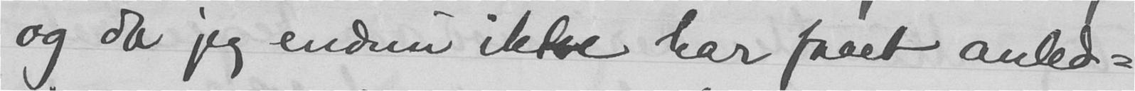og da jeg endnu ikke har faaet anled-
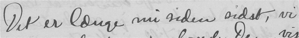Det er længe nu siden sidst, vi
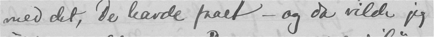med det, De havde faaet - og da vilde jeg
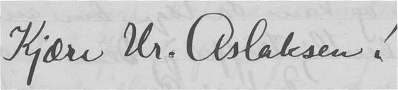Kjære Hr. Aslaksen!
